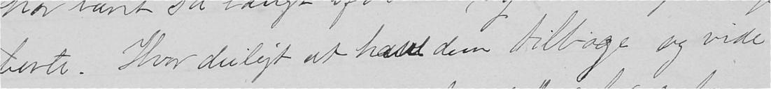borte. Hvor deiligt at have dem tilbage og vide
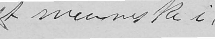ét menneske i
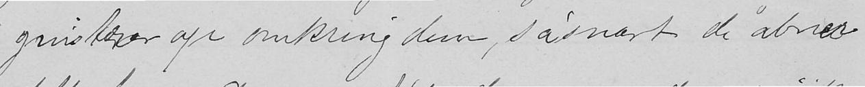gnistrer op omkring dem, såsnart de åbner
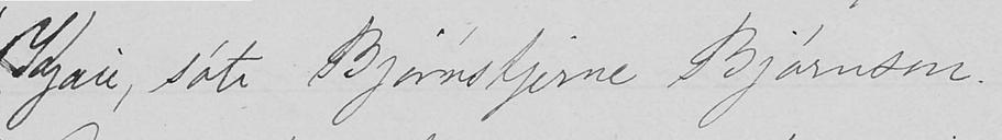Kjære, søte Bjørnstjerne Bjørnson.
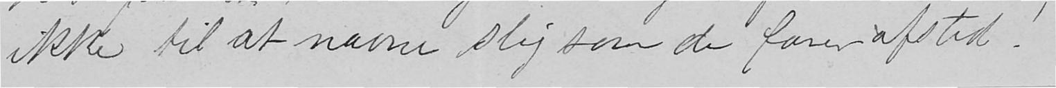ikke til at navne slig som de farer afsted!
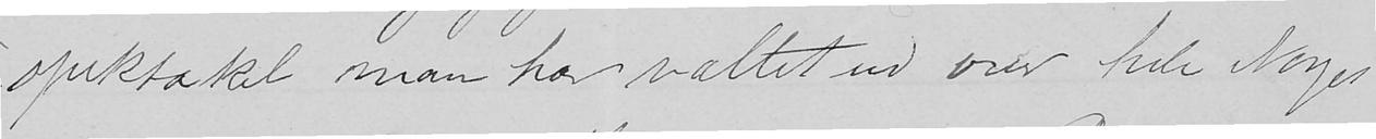spektakel man har valtet ud over hele Norges
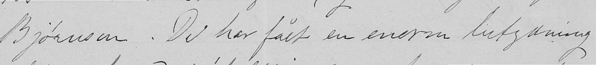Bjørnson. De har fået en enorn betydning
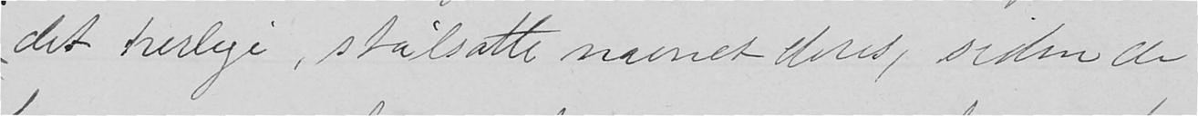det herlige, stålsatte navnet deres, siden de
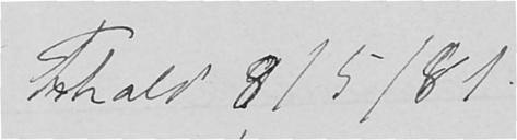Fr.hald 8/5/81.
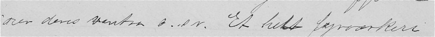over deres vantro o.s.v. Et helt fyrværkeri
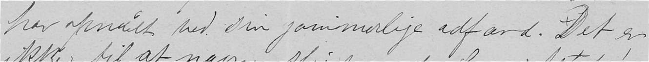har opnået ved sin jammerlige adfærd. Det er
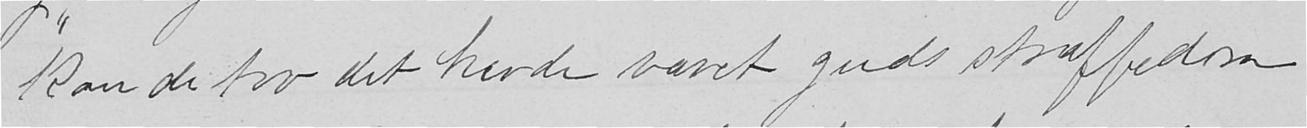kan de tro det havde været guds straffedom
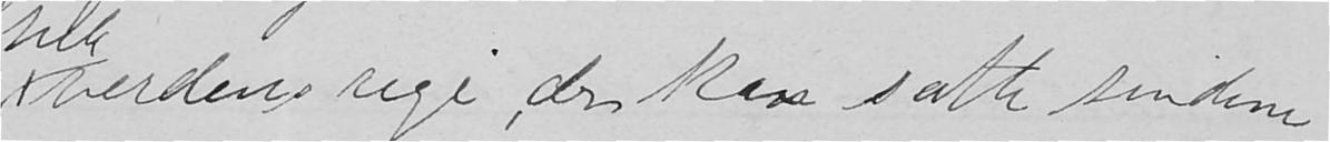verdens rige, der kan sætte sindene
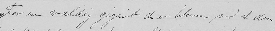For en vældig gigant de er bleven, ved al den
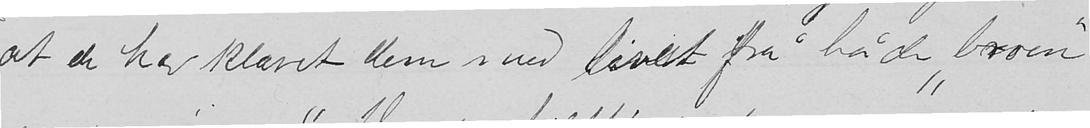at de har klaret dem med livet på både "broen"
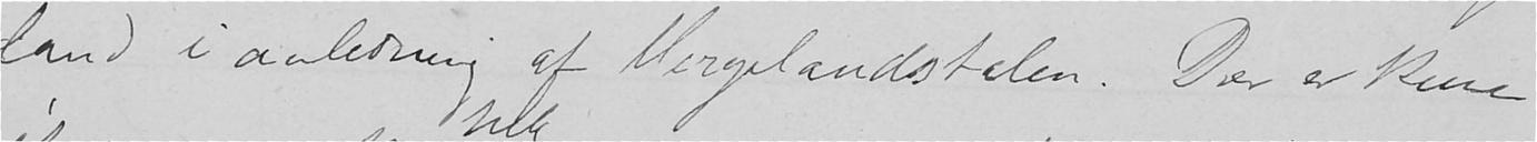land i anledning af Vergelandstalen. Der er kun
